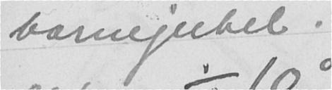barnejubel.
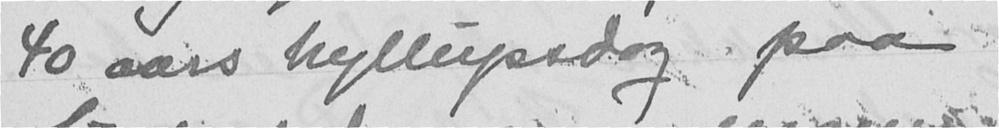40 aars bryllupsdag paa
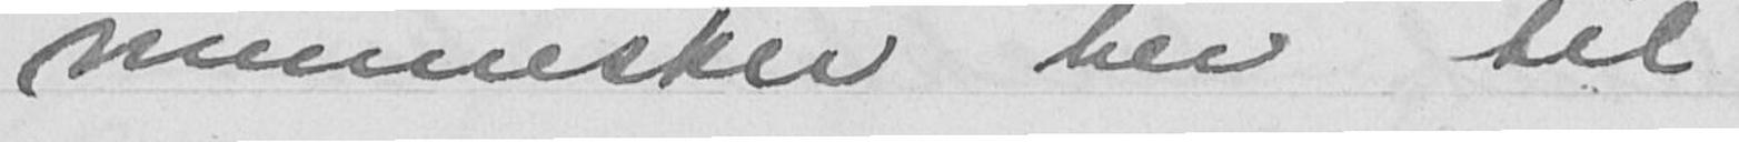mennesker her til
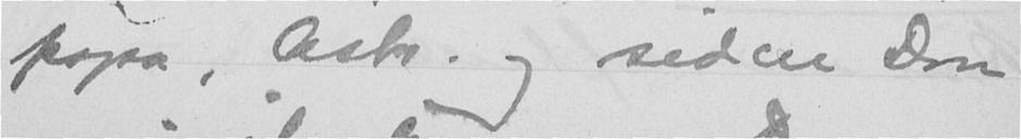papa, Astr. og siden Don
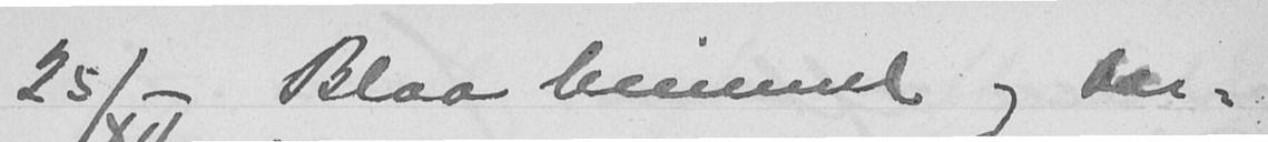25/XII Blaa himmel og bar-
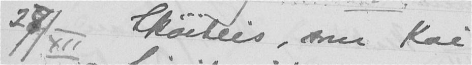28/XII Skøiteis, som Kai
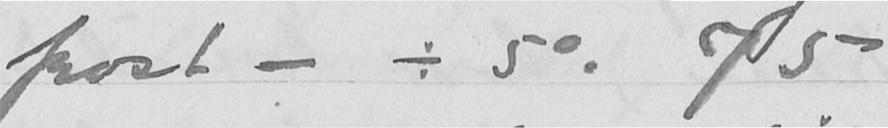frost - ÷ 5°. 75
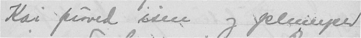Kai prøved isen og plumped
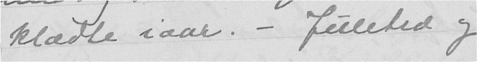klædte iaar. - Juletræ og
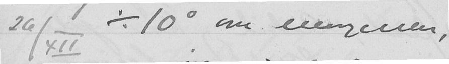26/XII ÷ 10° om morgenen,
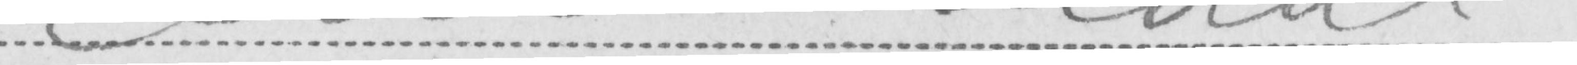Lille elvedal
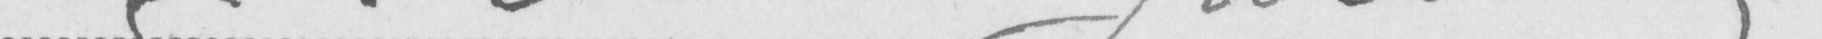Hr. Arne Garborg
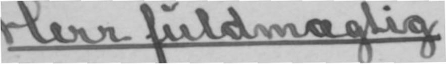Herr fuldmægtig
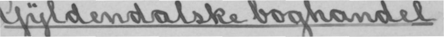Gyldendalske boghandel,
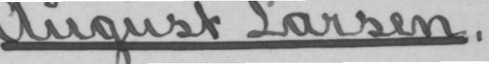August Larsen,
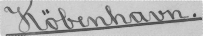København.
